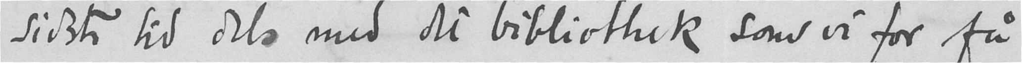sidste tid dels med det bibliothek som vi for få
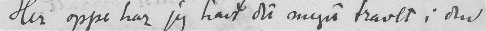Her oppe har jeg havt det meget travlt i den
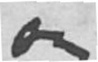og
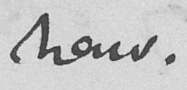han.
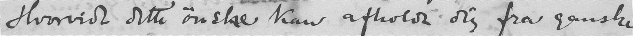Hvorvidt dette ønske kan afholde dig fra ganske
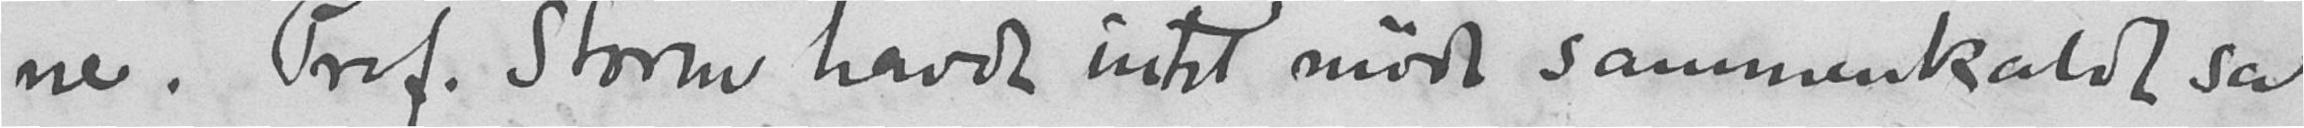ne. Prof. Storm havde intet møde sammenkaldt sa
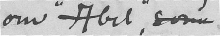om "Abel", som
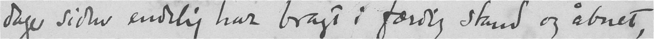dage siden endelig har bragt i færdig stand og åbnet,
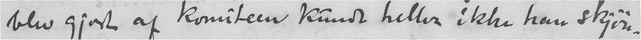blev gjort af komiteen kunde heller ikke han skjøn-
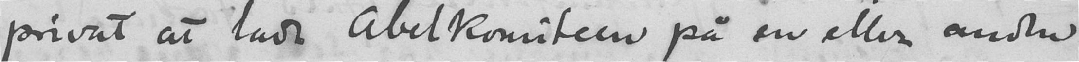privat at lade Abelkomiteen på en eller anden
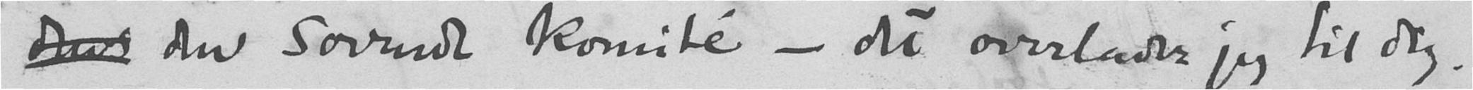dens den sovende komité - det overlader jeg til dig.
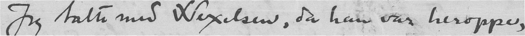Jeg talte med Wexelsen, da han var heroppe,
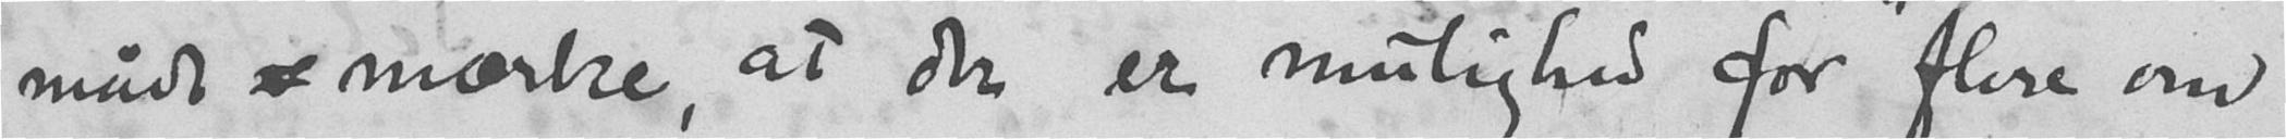måde mærke, at der er mulighed for "flere om
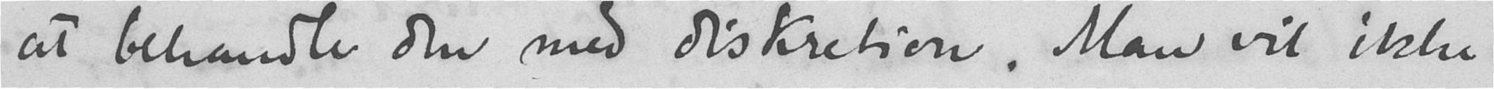at behandle den med diskretion. Man vil ikke
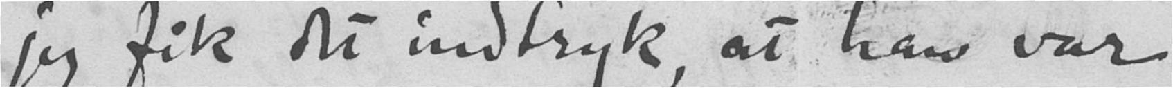jeg fik det indtryk, at han var
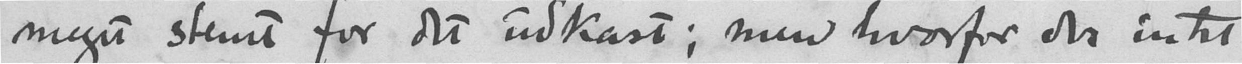meget stemt for det udkast; men hvorfor der intet
